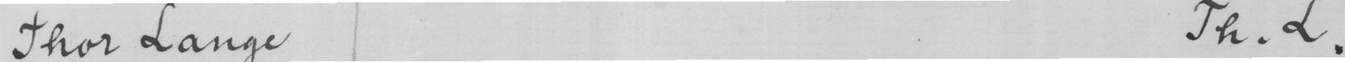Thor Lange Th.L.
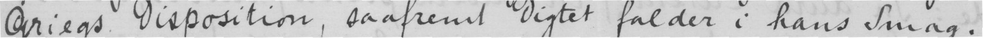Griegs Disposition, saafremt Digtet falder i hans Smag.
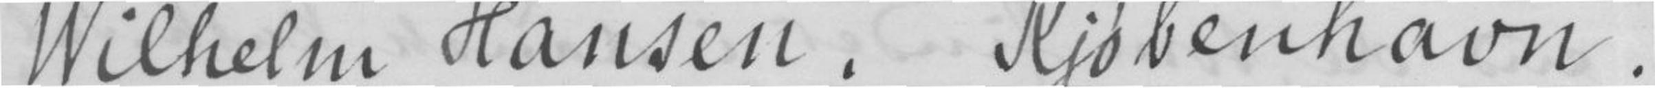Wilhelm Hansen, Kjøbenhavn
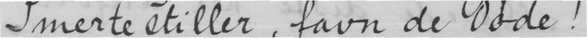Smertestiller, favn de Døde!
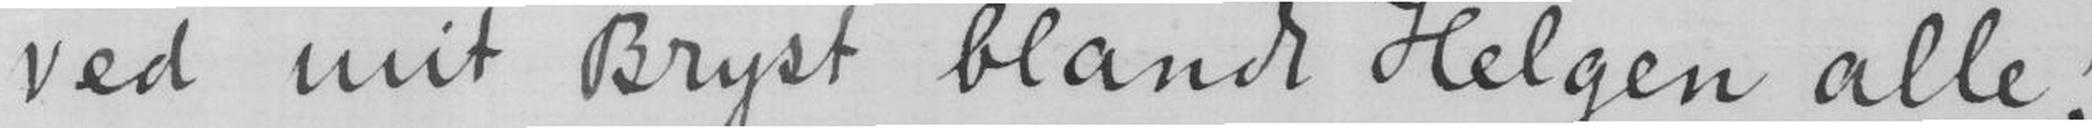ved mit Bryst blandt Helgen alle!
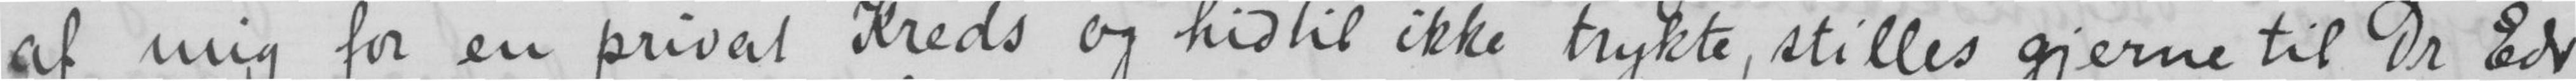af mig for en privat Kreds og hidtil ikke trykte, stilles gjerne til Dr. Edv.
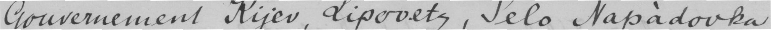Gouvernement Kijev, Lipovetz, Selo Napàdovka
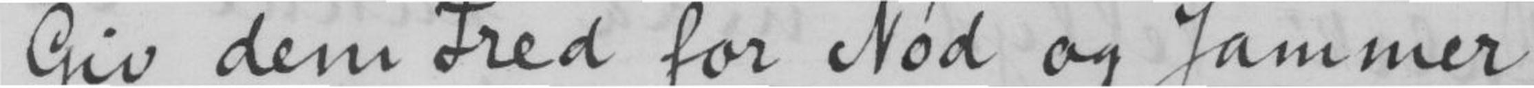Giv dem Fred for Nød og Jammer,
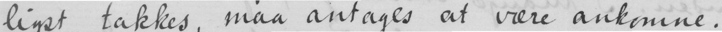ligst takkes, maa antages at være ankomne.
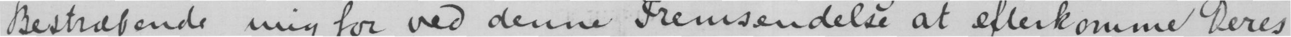Bestræbende mig for ved denne Fremsendelse at efterkomme Deres
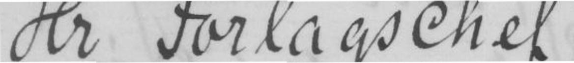Hr Forlagschef
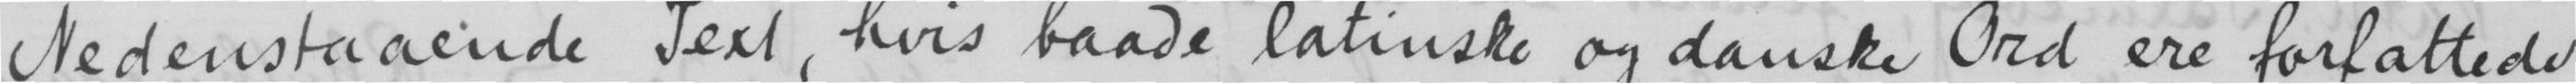Nedenstaaende Text, hvis baade latinske og danske Ord ere forfattede
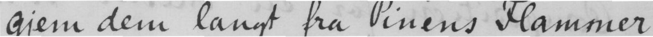gjem dem langt fra Piners Flammer
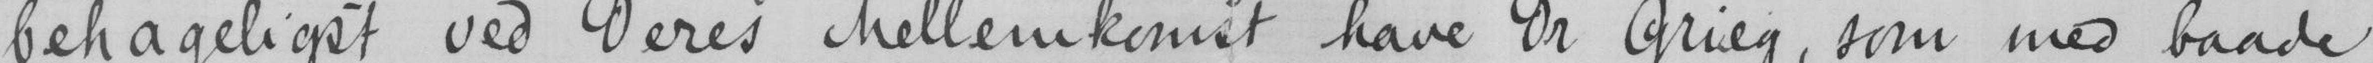behageligst ved Deres Mellemkomst have Dr. Grieg, som med baade
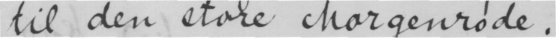til den store Morgenrøde.
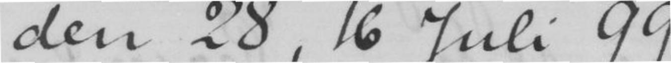den 28, 16 Juli 99
